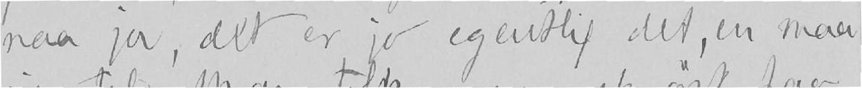naa ja, det er jo egentlig det, en maa
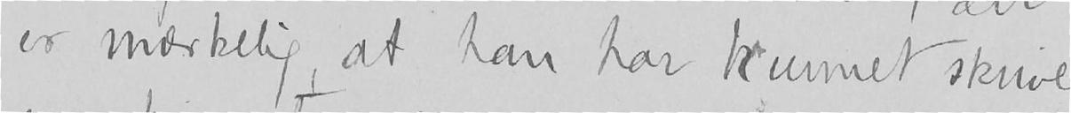er mærkelig, at han har kunnet skrive
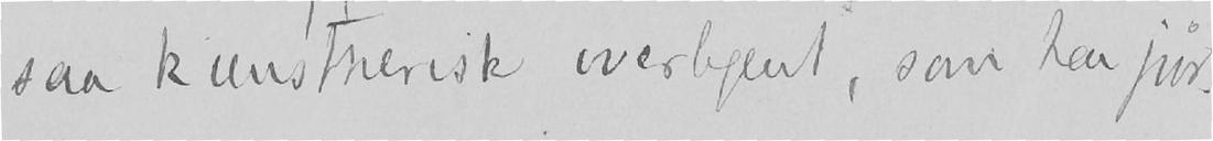saa kunstnerisk overlegent, som han giør.
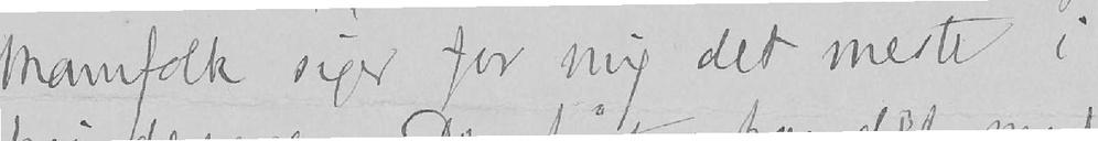Mannfolk siger for mig det meste i
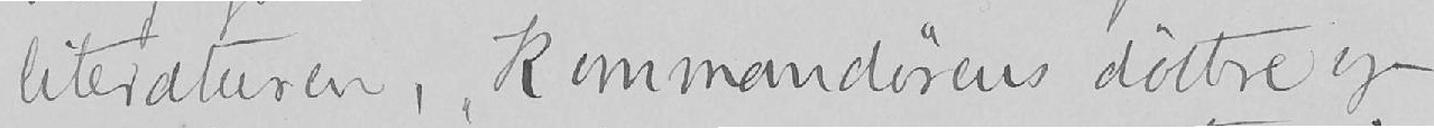literaturen, «Kommandørens døttre og
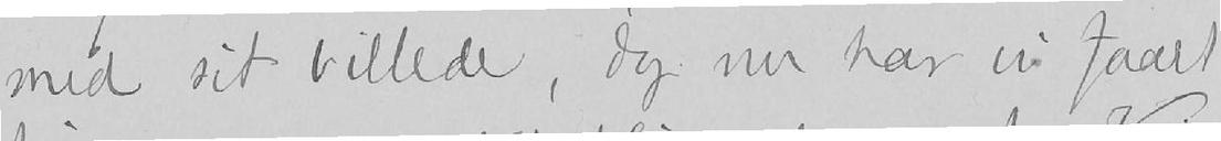med sit billede, dog nu har vi faaet
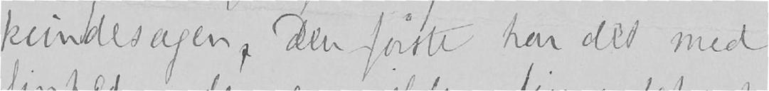kvindesagen. Den første har det med
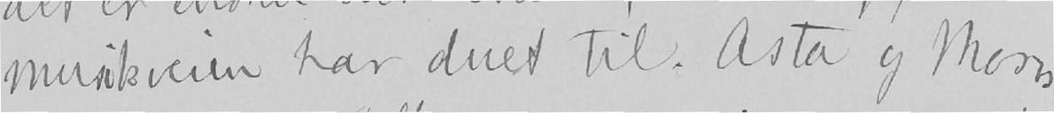musikveien har duet til. Asta og Mons
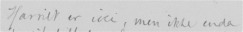Harriet er ivei, men ikke enda
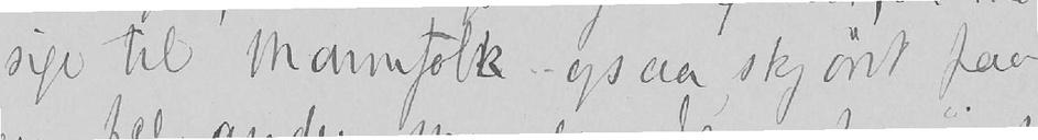sige til Mannfolk ogsaa, skjønt paa
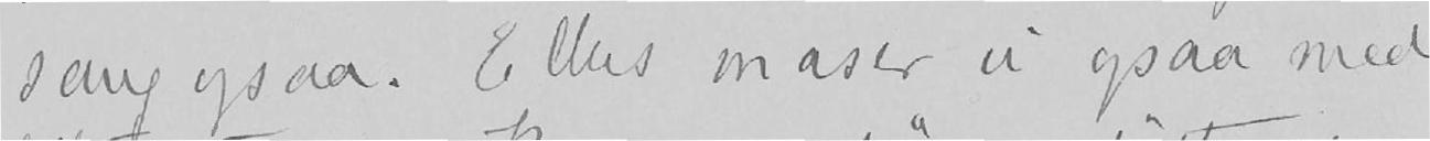sang ogsaa. Ellers maser vi ogsaa med
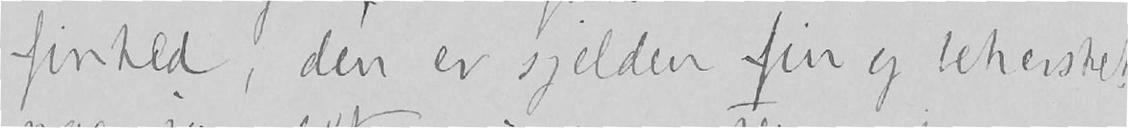finhed, den er sjelden fin og behersket,
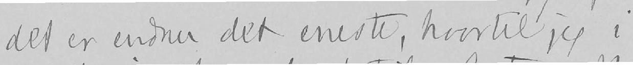det er endnu det eneste, hvortil jeg i
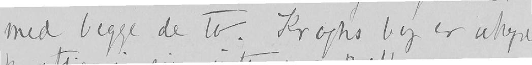med begge de to. Kroghs bog er uhyre
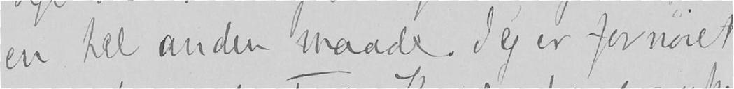en hel anden maade. Jeg er fornøiet
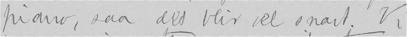piano, saa det blir vel snart. Vi
